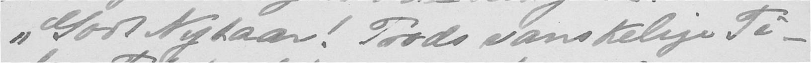"Godt Nytaar! Trods vanskelige Ti-
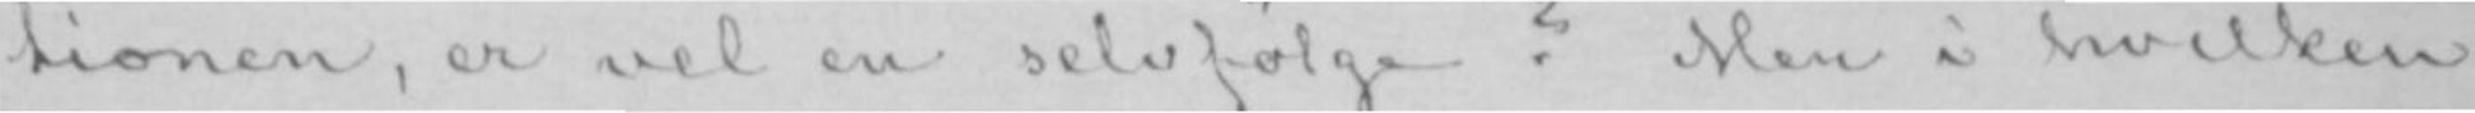tionen, er vel en selvfølge? Men i hvilken
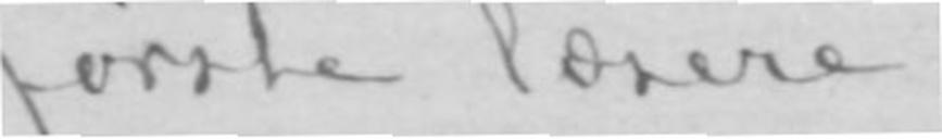første læsere.
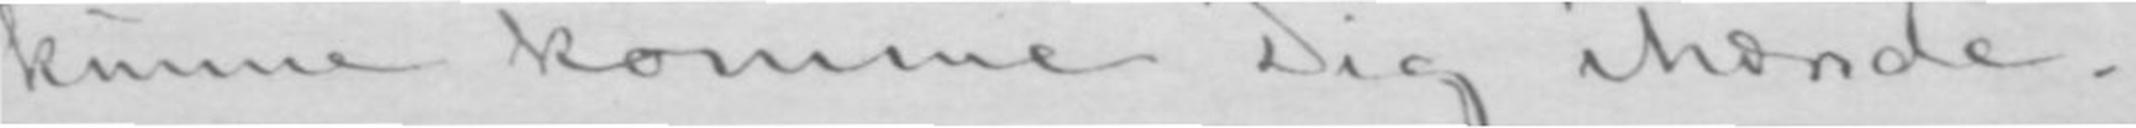kunne komme Dig ihænde.
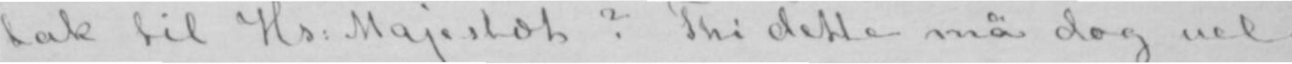tak til Hs: Majestæt? Thi dette må dog vel
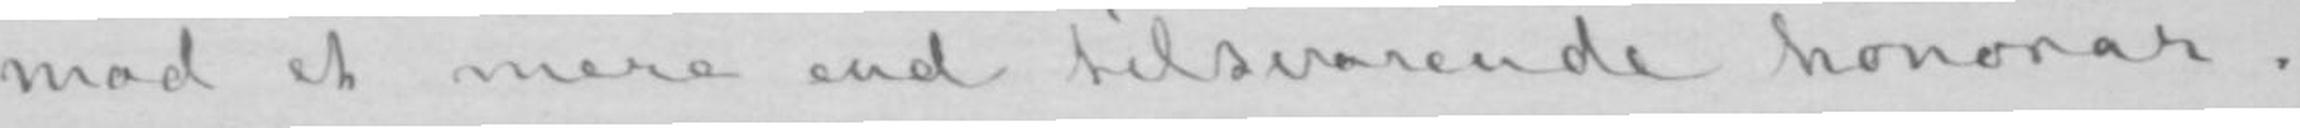mod et mere end tilsvarende honorar.
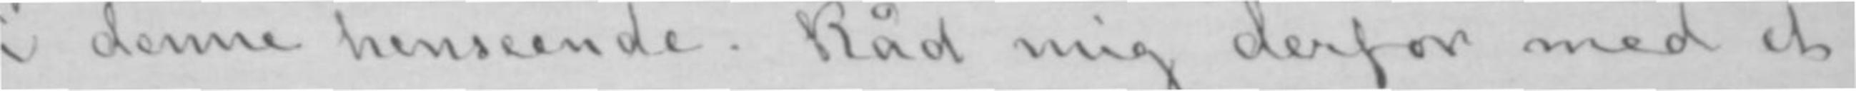i denne henseende. Råd mig derfor med et
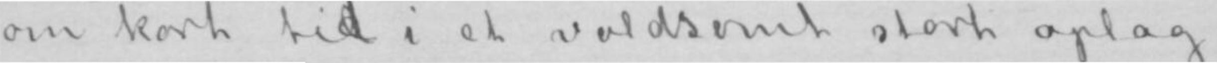om kort tidtil i et voldsomt stort oplag og
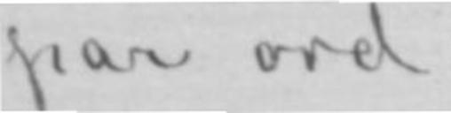par ord.
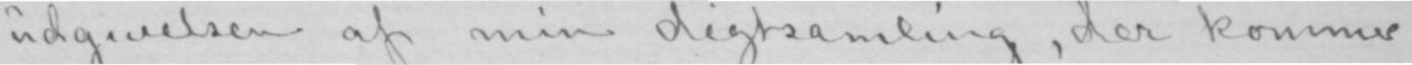udgivelsen af min digtsamling, der kommer
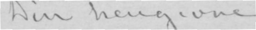Din hengivne
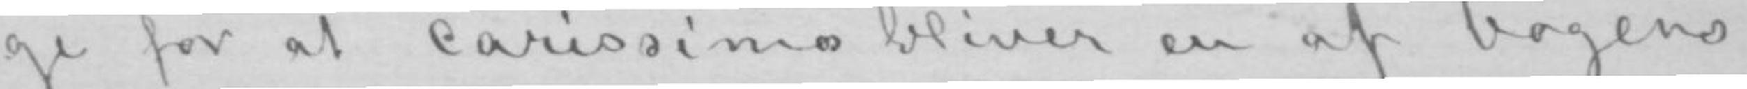ge for at carissimo bliver en af bogens
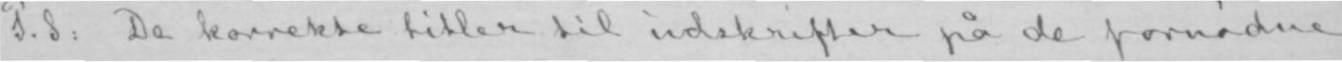P. S: De korrekte titler til udskrifter på de fornødne
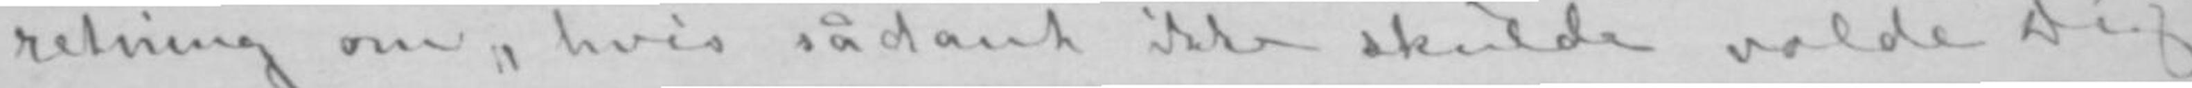retning om, hvis sådant ikke skulde volde Dig
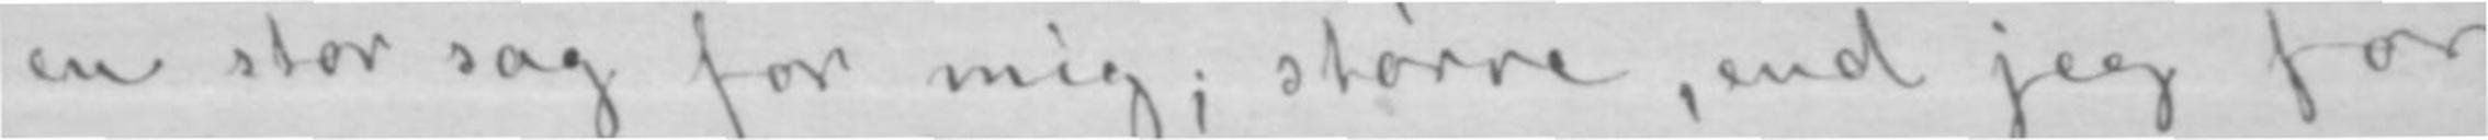en stor sag for mig; større, end jeg for
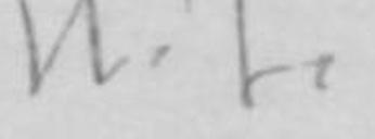H. I.
